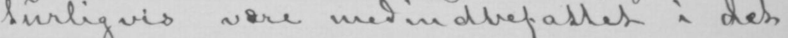turligvis være medindbefattet i det
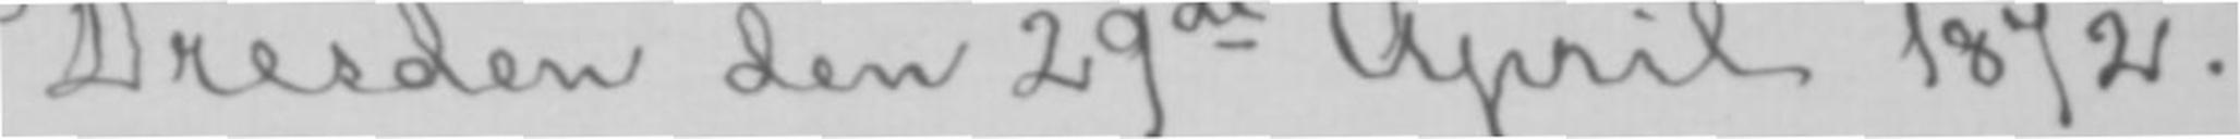Dresden den 29de April 1872.
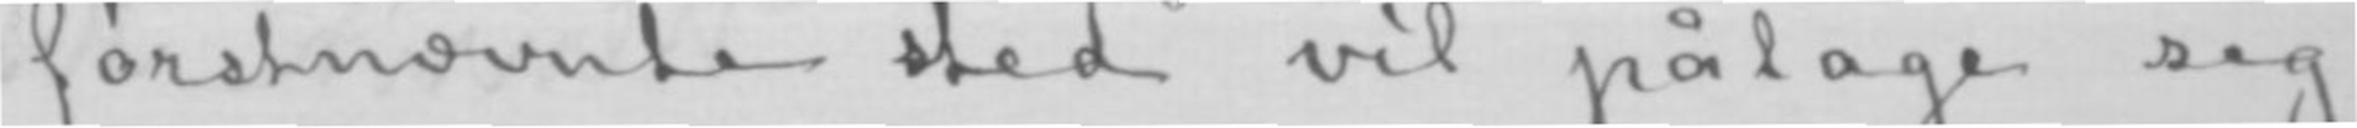førstnævnte sted vil påtage sig
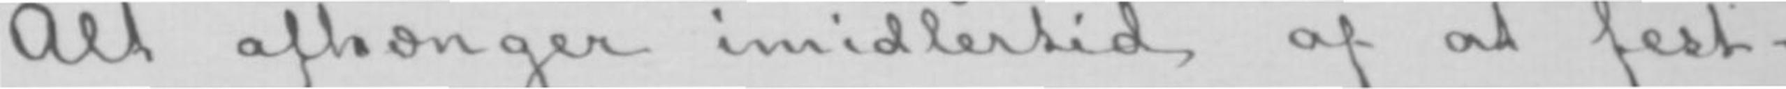Alt afhænger imidlertid af at fest-
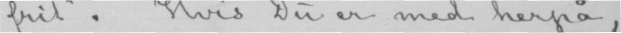frit». Hvis Du er med herpå,
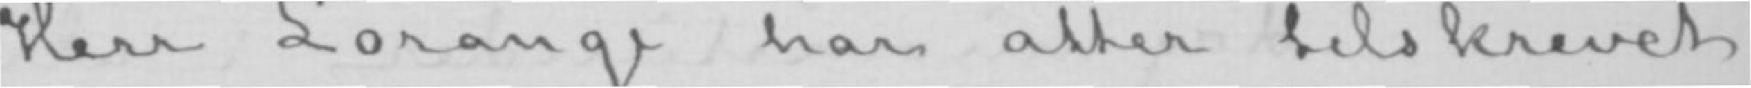Herr Lorange har atter tilskrevet
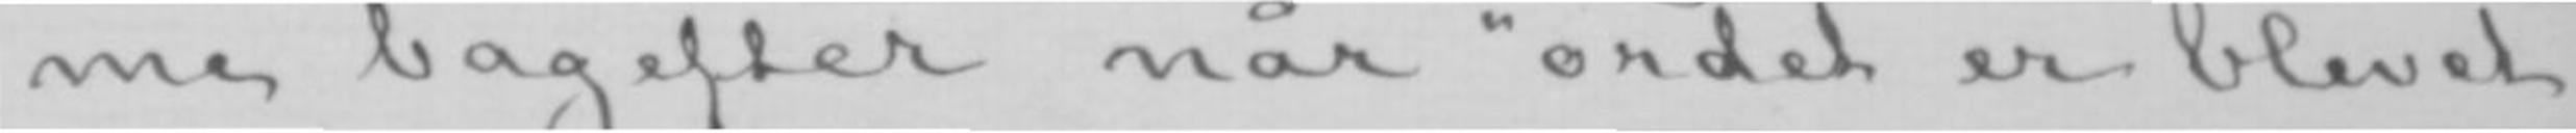me bagefter når «ordet er blevet
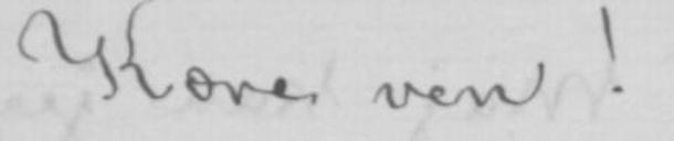Kære ven!
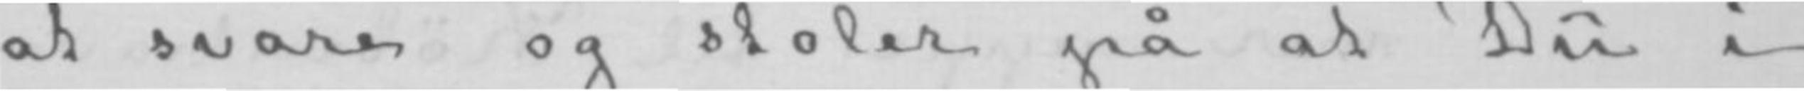at svare og stoler på at Du i
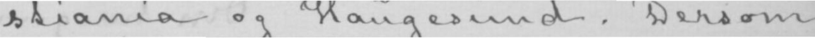stiania og Haugesund. Dersom
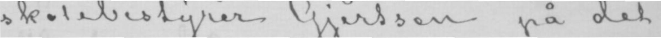skolebestyrer Gjertsen på det
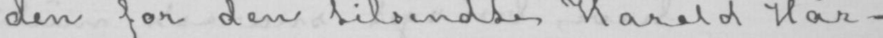den for den tilsendte Harald Hår-
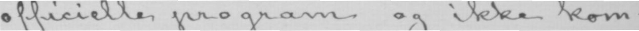officielle program og ikke kom-
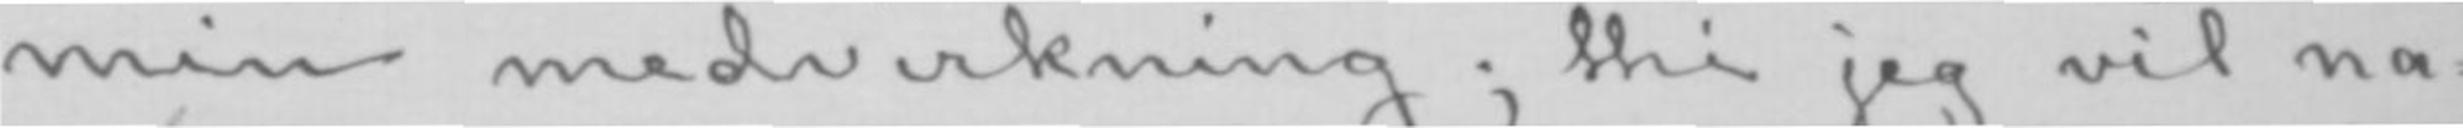min medvirkning; thi jeg vil na-
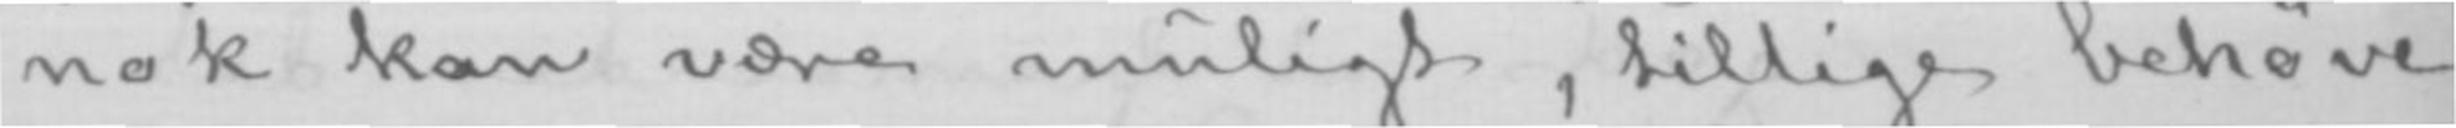nok kan være muligt, tillige behøve
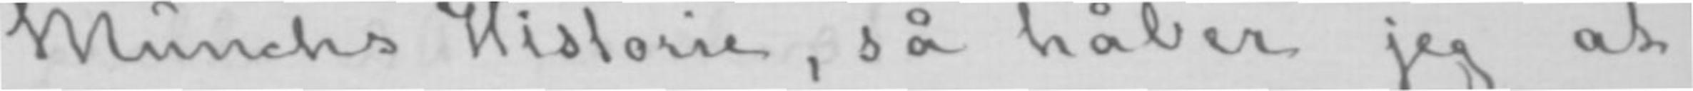Munchs Historie, så håber jeg at
